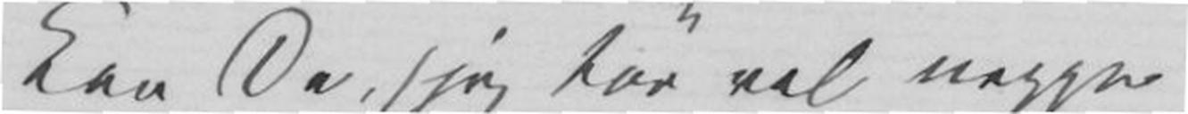Kan De, (jeg tør vel neppe
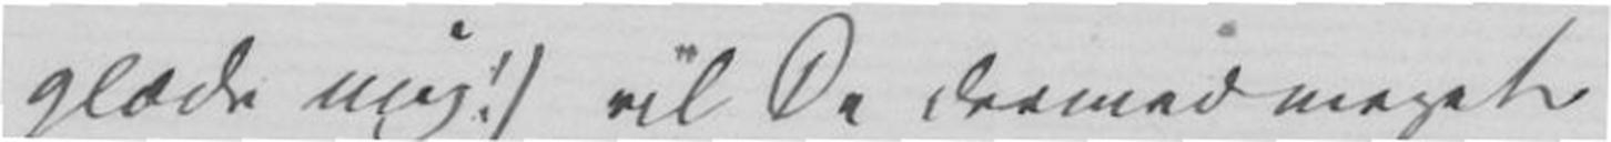glæde mig!) vil De dermed meget
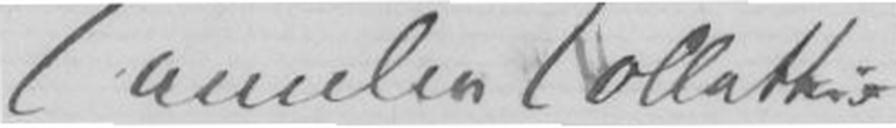Camilla Collet
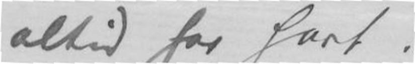altid har havt.
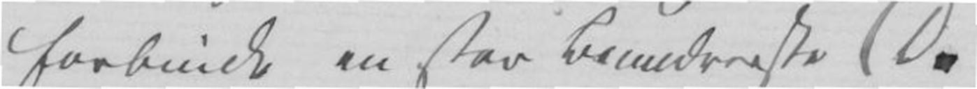forbinde en stor beundrerske De
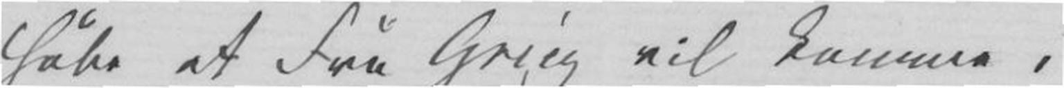håbe at Fru Grieg vil komme i
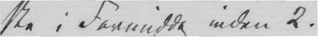ske i Formiddag inden 2.
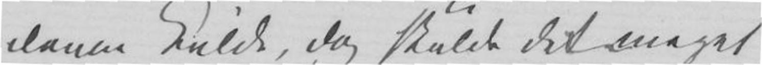denne Kulde, dog skulde det meget
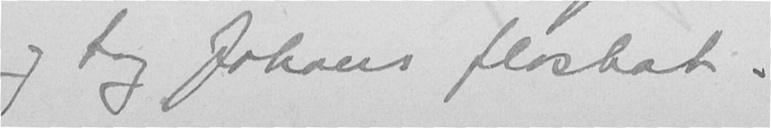og tog Johans floshat.
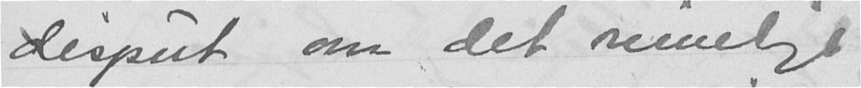disput om det rimelige
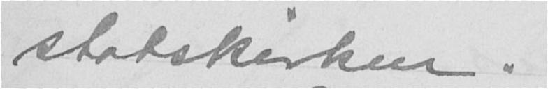statskirken.
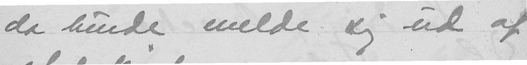da kunde melde sig ud af
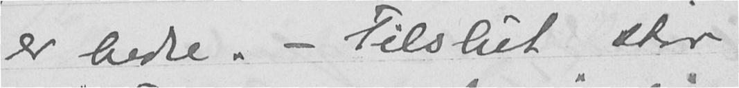er bedre. - Tilslut stor
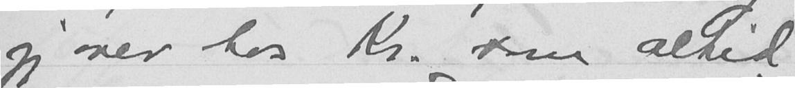jeg over hos Kr. som altid
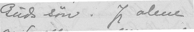Guds søn. Jeg alene
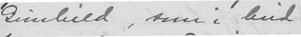Gunhild, som i hvid
